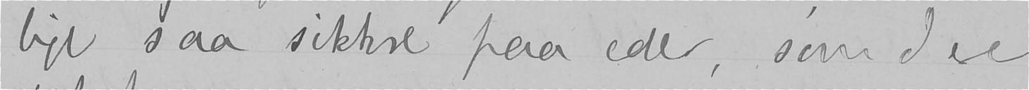lige saa sikkre paa eder, som dere
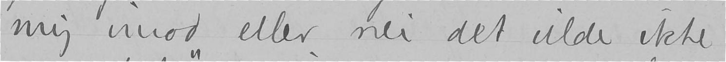mig imod eller nei det vilde ikke.
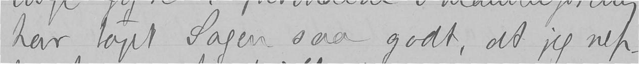har taget Sagen saa godt, at jeg nep-
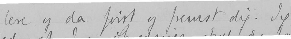lere og da først og fremst dig. Jeg
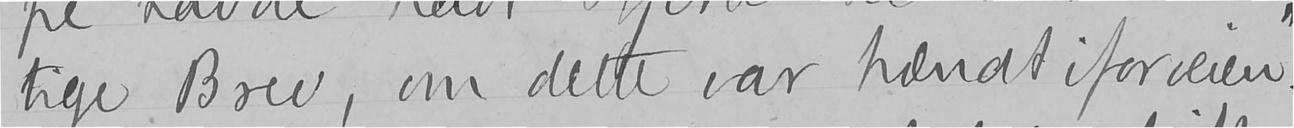tige Brev, om dette var hændt iforveien."
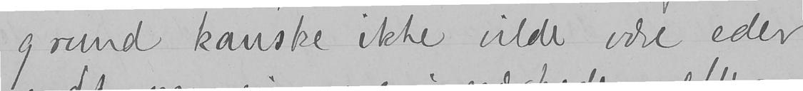grund kanske ikke vilde være eder
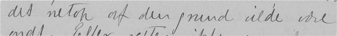det netop af den grund vilde være
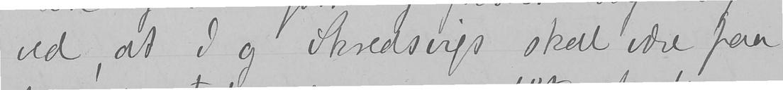ved at I og Skredsvigs skal være paa
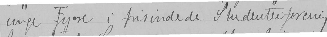unge Fyre i frisindede Studenterforening
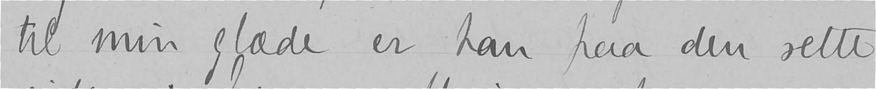til min glæde er han paa den rette
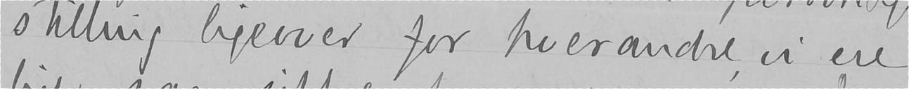stilling ligeover for hverandre, vi ere
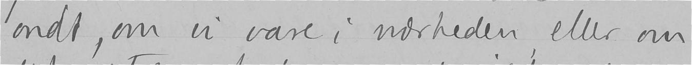ondt, om vi vare i nærheden, eller om
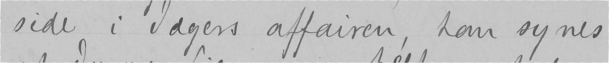side i Jægers affairen, han synes
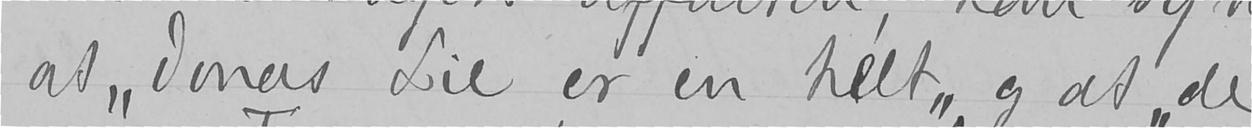at "Jonas Lie er en helt" og at "de
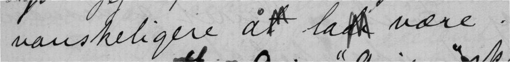vanskeligere åt la være.
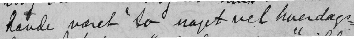havde været "to noget vel hverdags-
